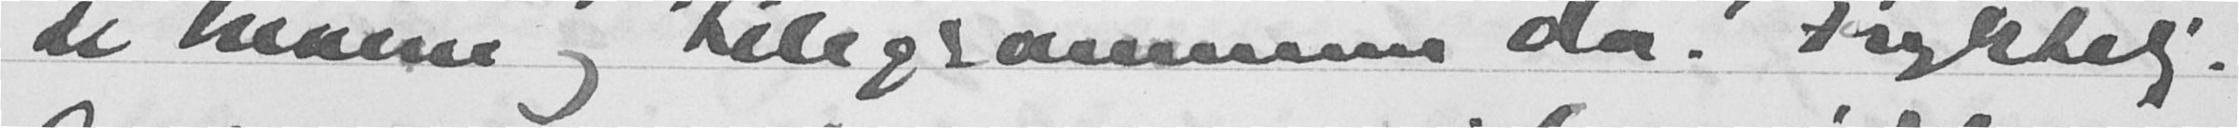de brevene og telegrammene da! Fryktelig.
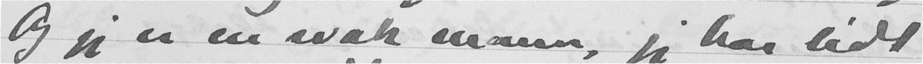Og jeg er en svak mann, jeg har lidt
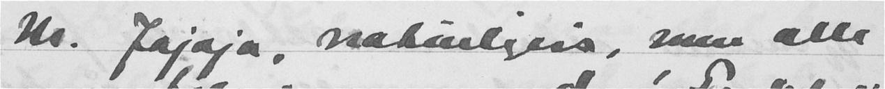M: Jajaja, naturligvis, men alle
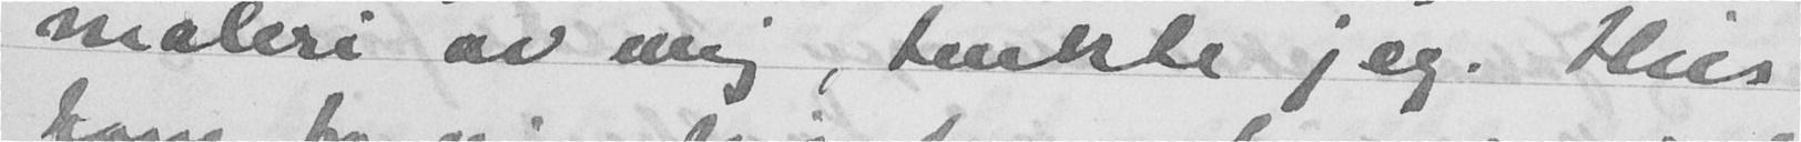maleri av mig, tenkte jeg. Hils
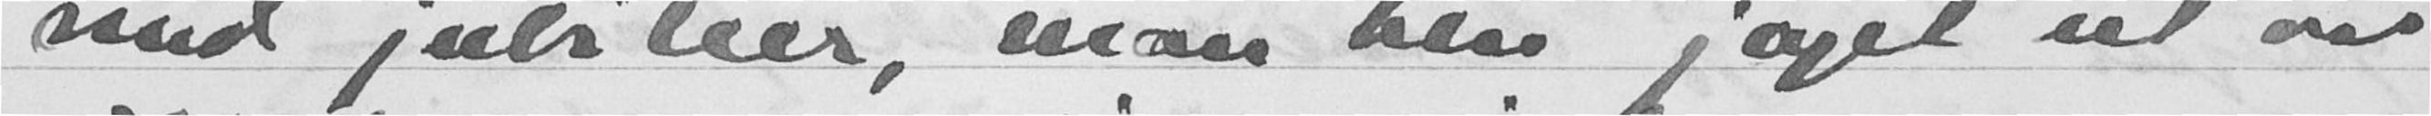med jubileer, man blir jaget ut av
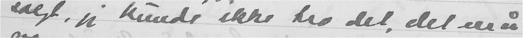solgt, jeg kunde ikke tro det, det må
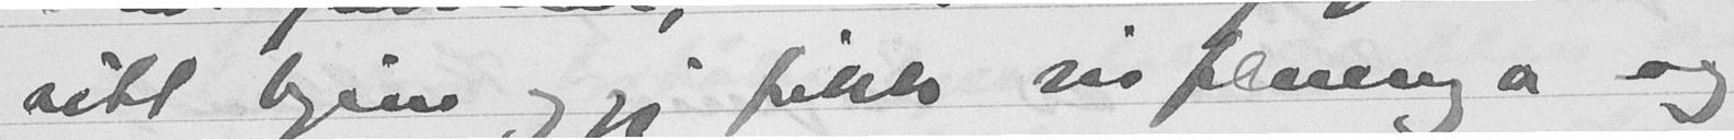sitt hjem og jeg fikk influenza og
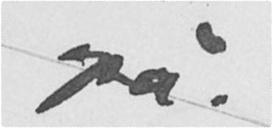også.
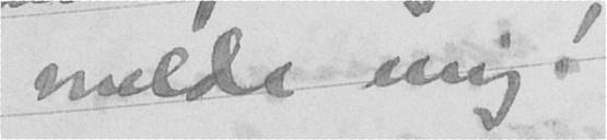melde mig!
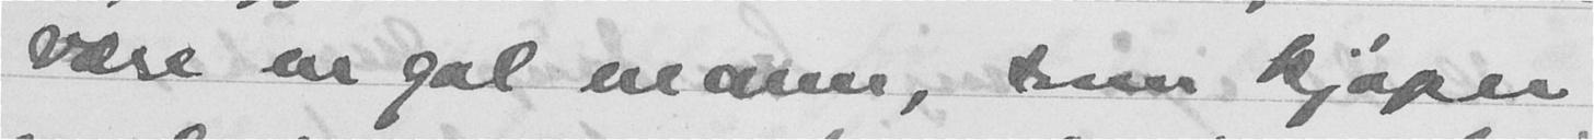være en gal man, som kjøper
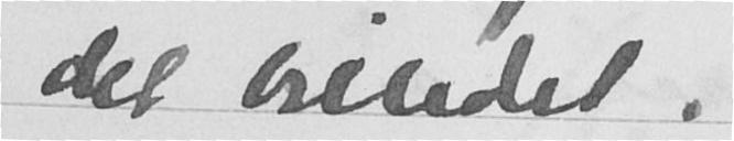det billedet.
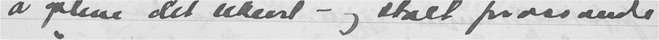å opleve det likevel - og stort for oss andre
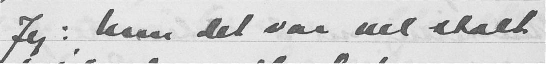Jeg: men det var vel stort
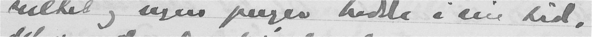sultet og ingen penger hadde i min tid,
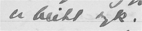er blitt syk.
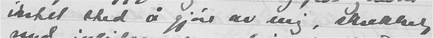intet sted å gjøre av mig, skrekkelig
